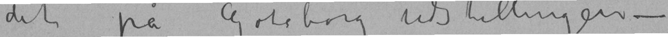det på Göteborg udstillingen –
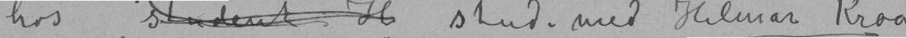hos student H stud. med Hilmar Krog
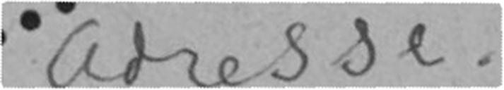Adresse.
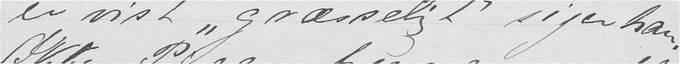er vist "græsseligt" siger han.
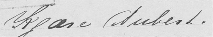Kjære Aubert.
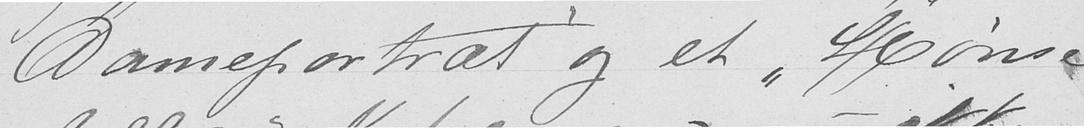Dameportræt" og et "Hønse-
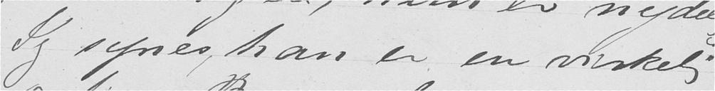Jeg synes han er en virkelig
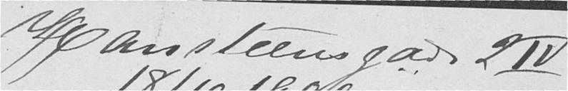Hansteens gade 2 IV
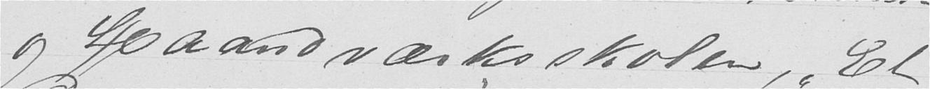og Haandværksskolen, "Et
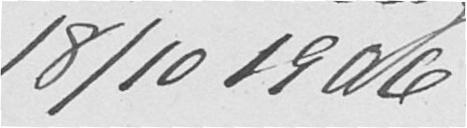18/10 1906
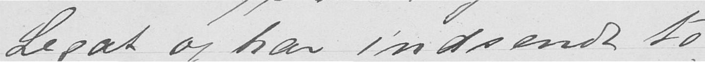Legat og har indsendt to
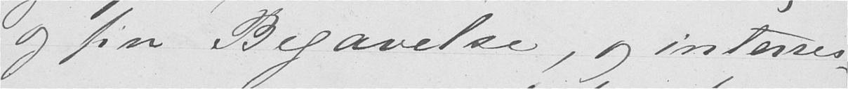og fin Begavelse, og interres-
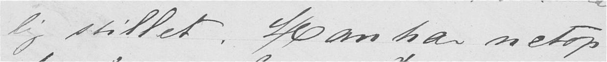lig stillet. Han har netop
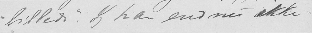billede". Jeg har endnu ikke
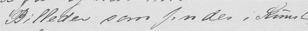Billeder som findes i Kunst-
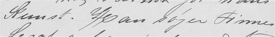Kunst. Han søger Times
